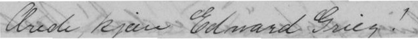Ærede kjære Edvard Grieg!
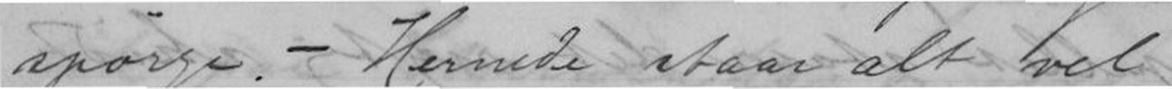spørge. - Hernede staar alt vel
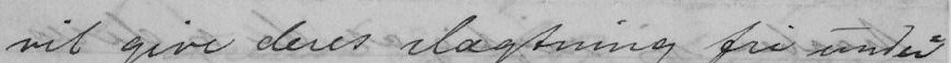vil give deres slægtning fri under-
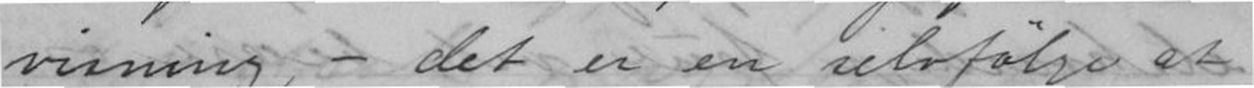visning, - det er en selvfølge at
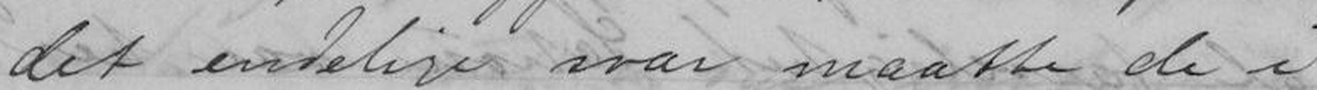det endelige svar maatte de i
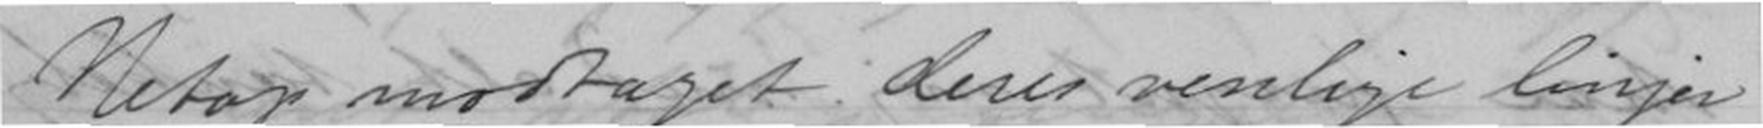Netop modtaget deres venlige linjer,
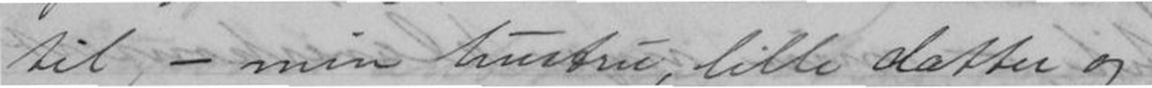til, - min hustru, lille datter og
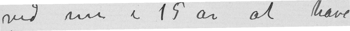ved nu i 15 år at have
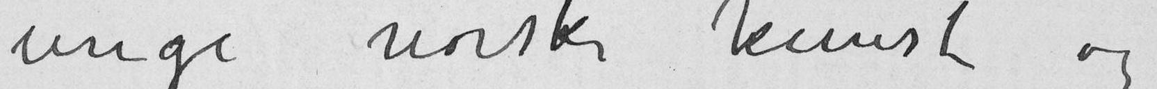unge norske kunst og
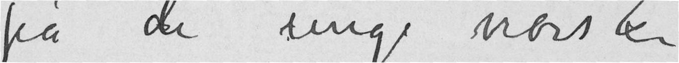på de unge norske
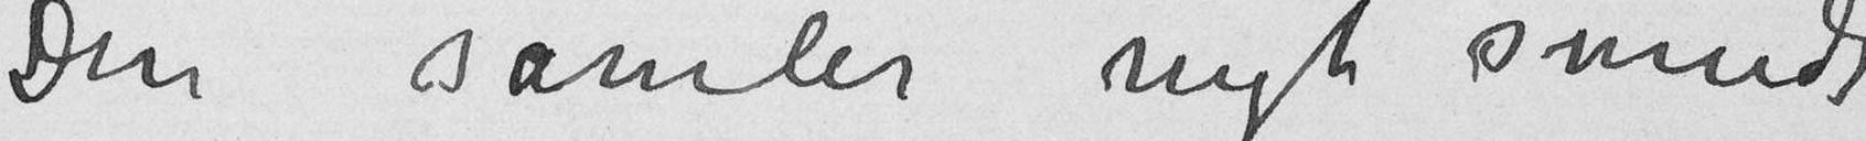Den samler nyt smuds
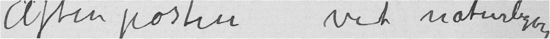Aftenposten vet naturligvis
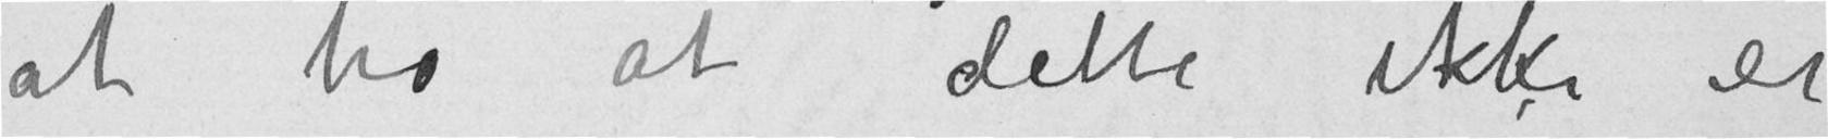at tro at dette ikke er
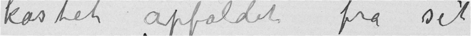kastet affaldet fra sit
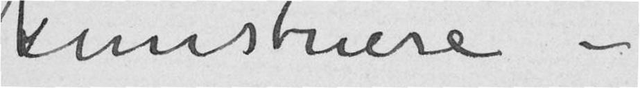kunstnere –
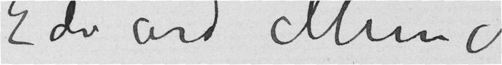Edvard Munch
